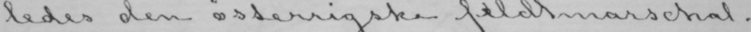ledes den østerrigske feldtmarschal-
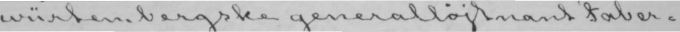würtembergske generalløjtnant Faber-
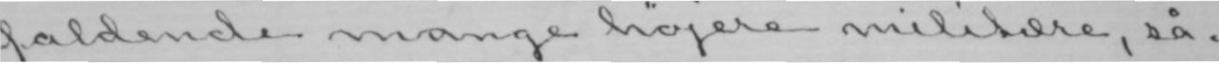faldende mange højere militære, så-
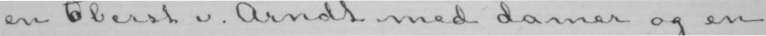en Ooberst v. Arndt med damer og en
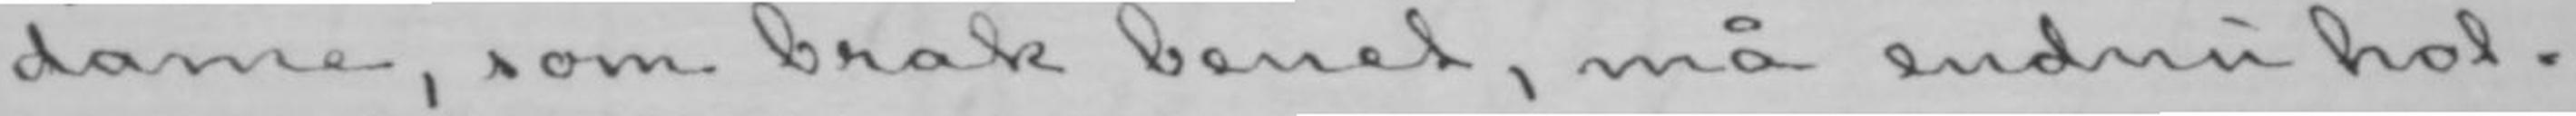dame, som brak benet, må endnu hol-
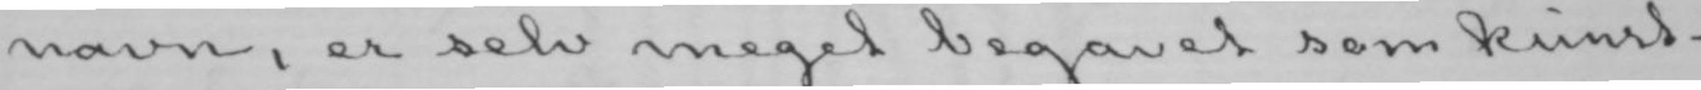navn, er selv meget begavet som kunst-
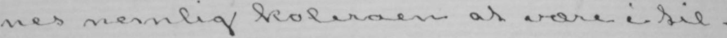nes nemlig koleraen at være i til-
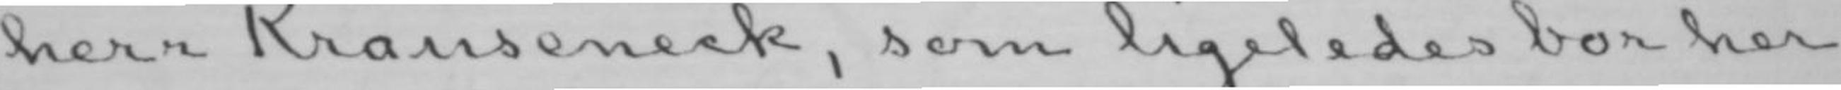herr Krauseneck, som ligeledes bor her
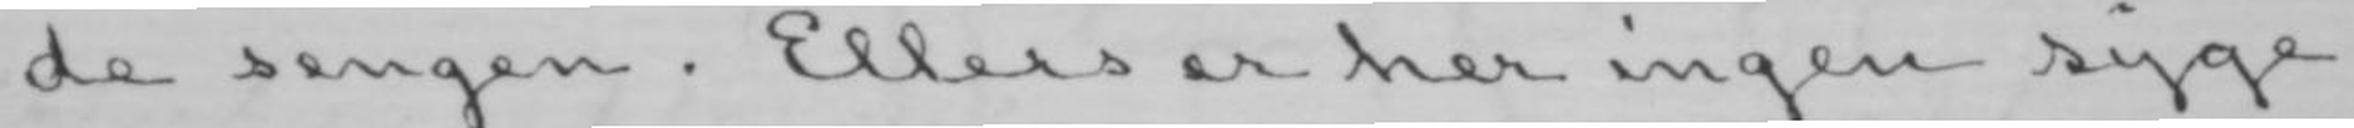de sengen. Ellers er her ingen syge.
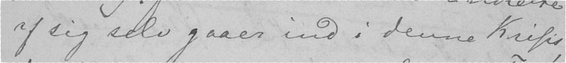af sig selv gaaer ind i denne Krisis,
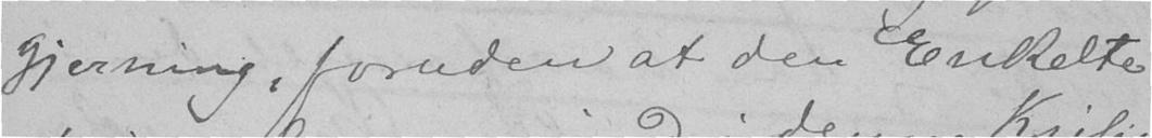gjerning, foruden at den Enkelte
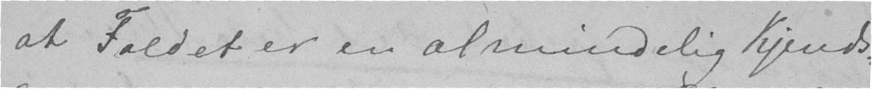at Faldet er en almindelig Kjends-
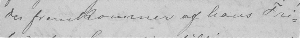der fremkommer af hans Fri-
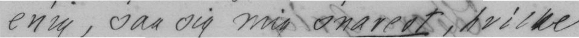enig, saa sig mig snarest, hvilke
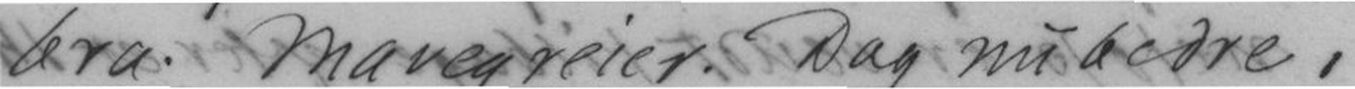bra. Mavegreier. Dog nu bedre,
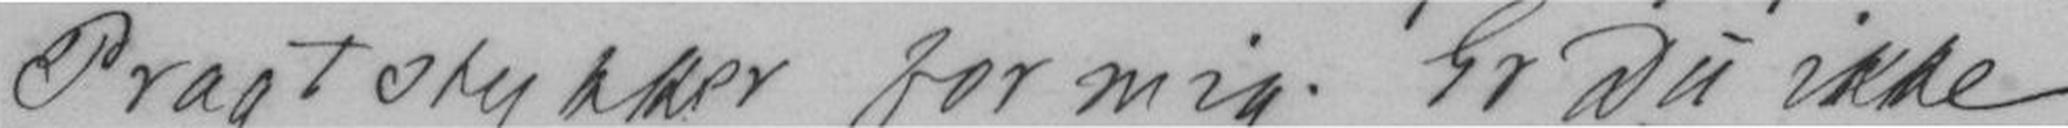Bragt stykker for mig. Er Du ikke
